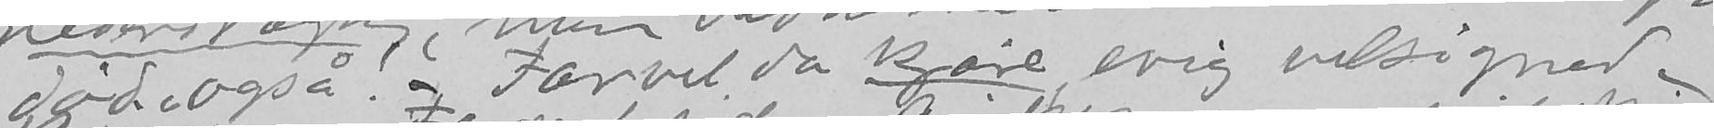død også!- Farvel da kjære evig velsignede
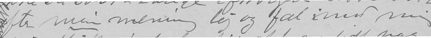efter min mening lej og fæl imod mig
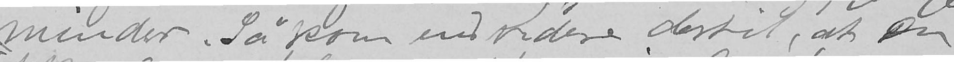minder. Så kom end end videre dertil, at Du
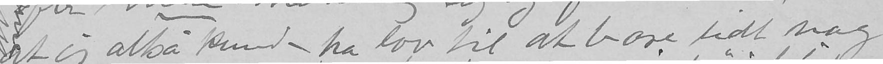at jeg altså kunde ha lov til at bære lidt nag
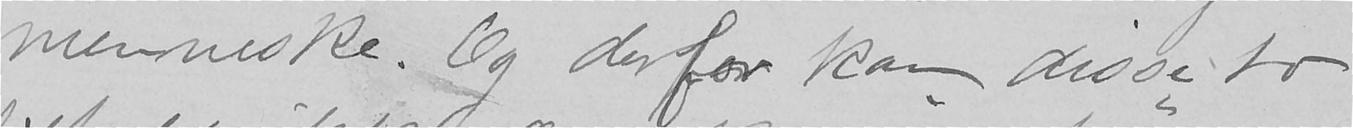menneske. Og derfor kan disse to
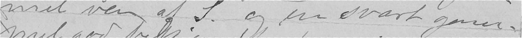mel ven at S. og en svært gam-
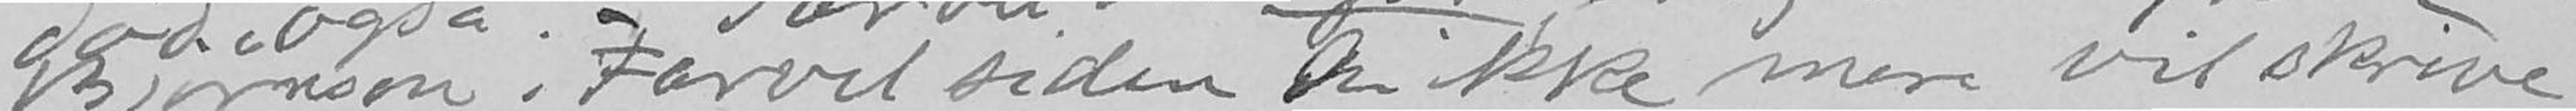Bjørnson. Farvel siden Du ikke mere vil skrive
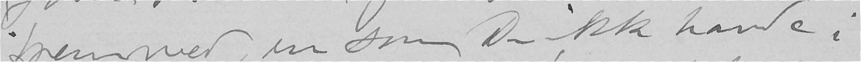fremmed, en som de ikke havde i
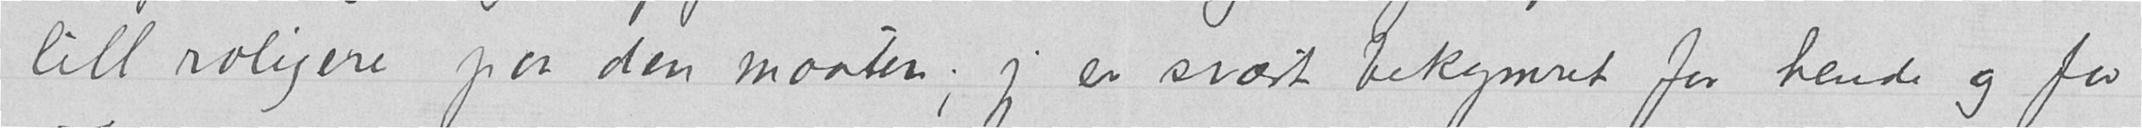litt roligere paa den maaten; jeg er svært bekymret for hende og for
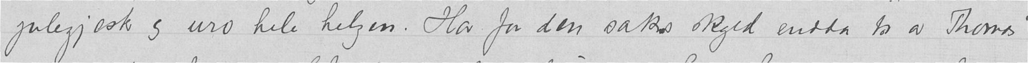julegjester og uro hele helgen. Har for den saks skyld endda to av Thomas’
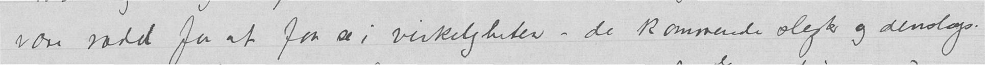være rædd for at faa se i virkeligheten - de kommende slegter og denslags.
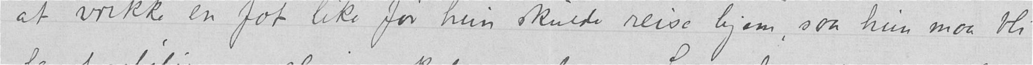at vrikke en fot like før hun skulde reise hjem, saa hun maa bli
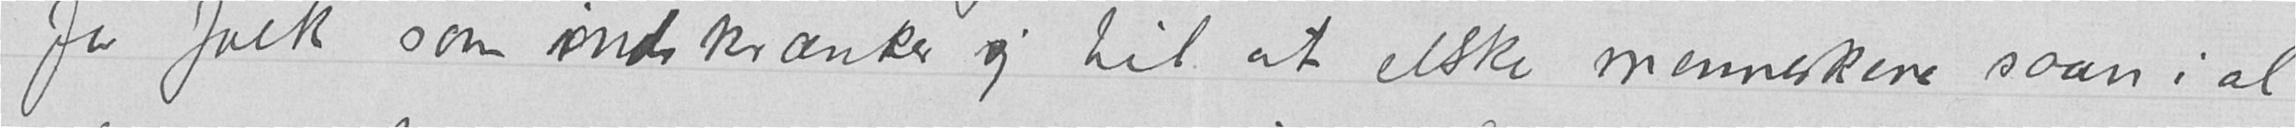for folk som indskrænker sig til at elske menneskene saan i al
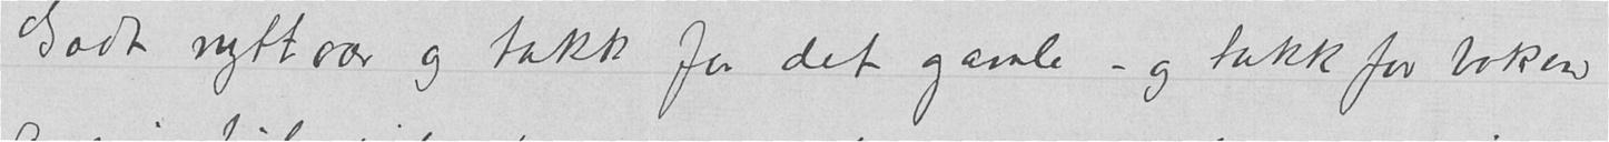Godt nyttaar og takk for det gamle – og takk for boken
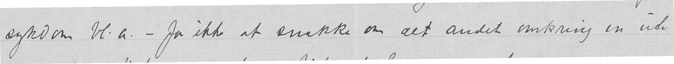sykdom bl.a. – for ikke at snakke om alt andet omkring en ute
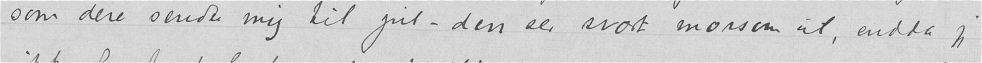som dere sendte mig til jul – den ser svært morsom ut, endda jeg
